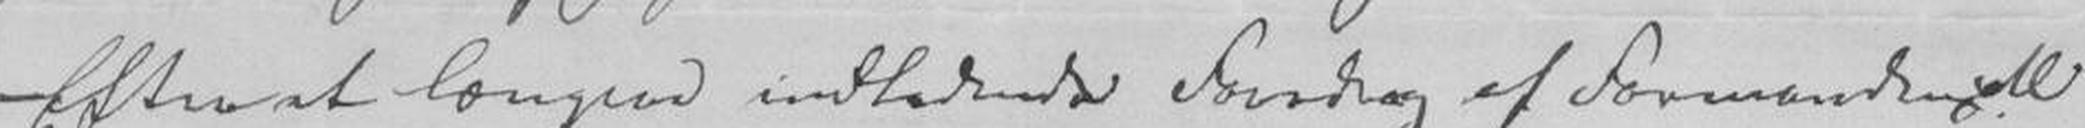Efter et længere indladende Foredrag af Formanden M
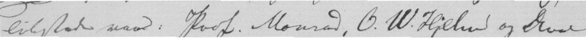Tilstede var: Prof. Monrad, O. W.Hjelm og Deres
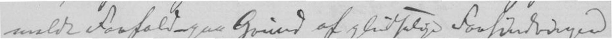meldt forfald paa Grund af pludselige Forhindringer
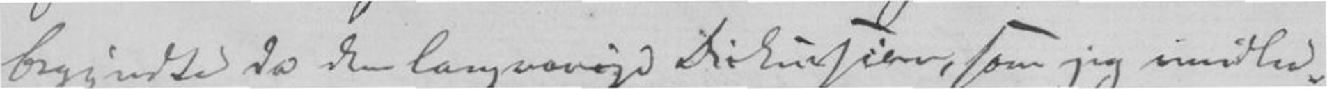begyndte da den langvarige Diskussion, som jeg imidler
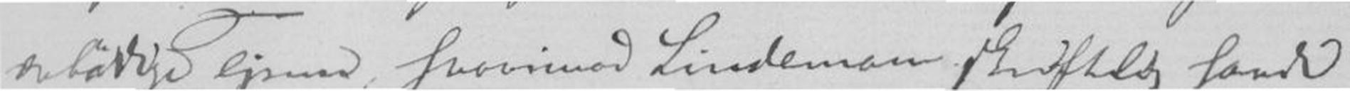ærbødige Tjener, hvorimod Lindeman skriftlig havde
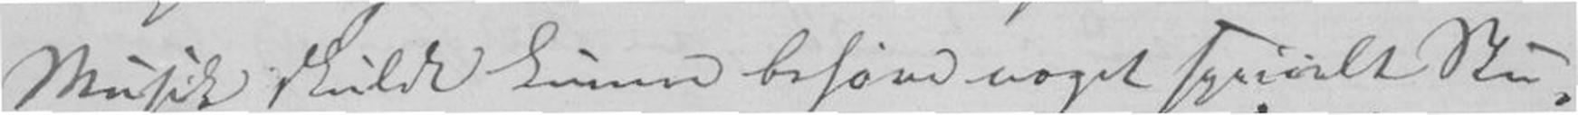Musik skulde kunne behøve noget spesielt Stu-
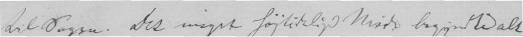til Sagen. Det meget højtidlige Møde begyndte alt
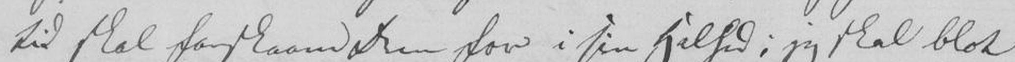tid skal forskaane Dem for i sin Helhet; jeg skal blot
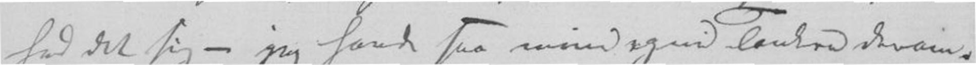hed det sig - jeg havde saa mine egne Tanker derom.
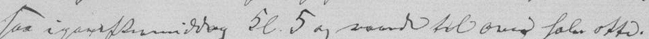saa igaareftermiddag kl 5 og varede til over halv otte.
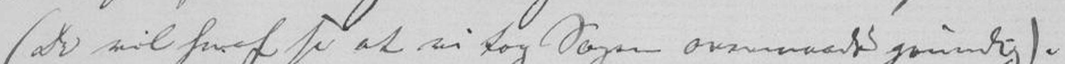(De vil heraf se af vi tog Sagen overmaade grundig).
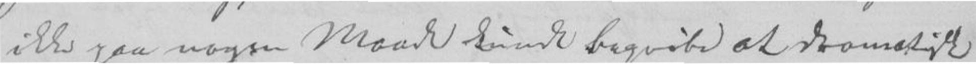ikke paa nogen Maade kunde begribe at dramatisk
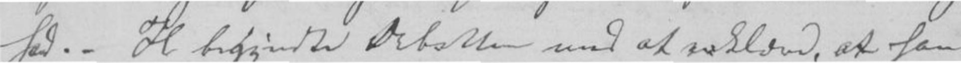hed. - H. begyndte Debatten med at erklære, at han
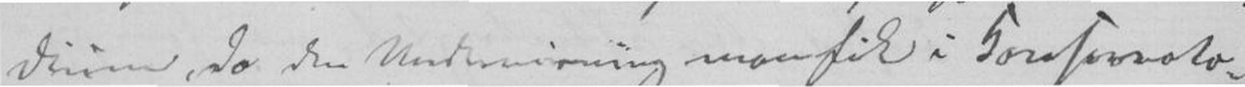dium, da den Undervisning man fik i Konservato-
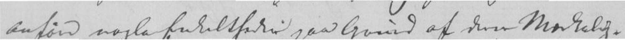anføre nogle Enkeltheder paa Grund af denne Mærkelig
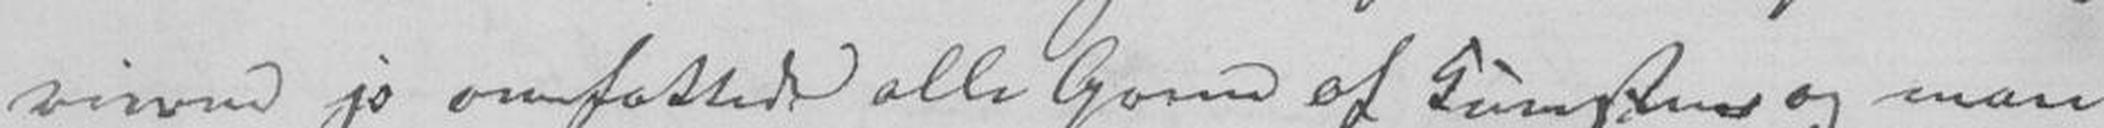rium jo omfattede alle G... af Kunsten og man
